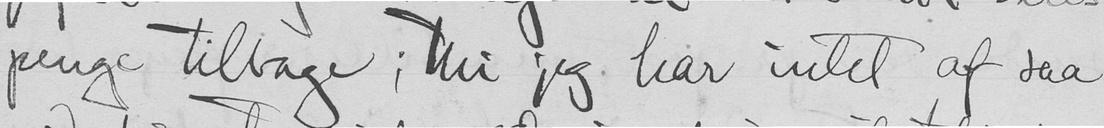penge tilbage; thi jeg har intet af saa
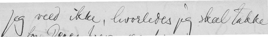Jeg veed ikke, hvorledes jeg skal takke
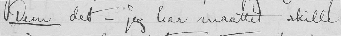Dem det - jeg har maattet skillle
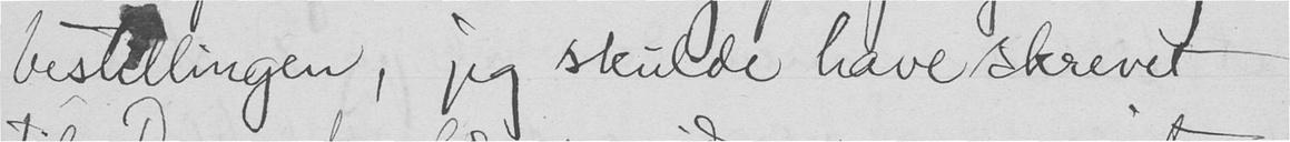bestillingen, jeg skulde have skrevet
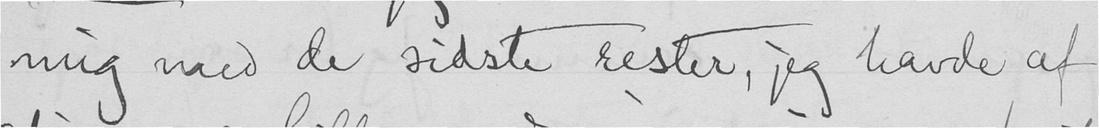mig med de sidste rester, jeg havde af
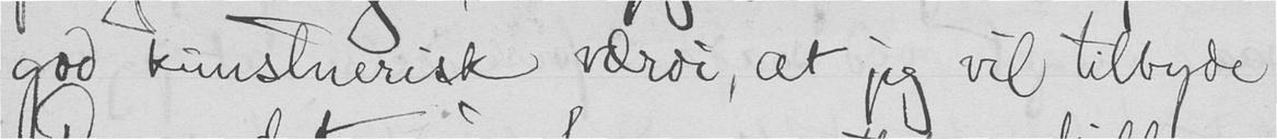god kunstnerisk værdi, at jeg vil tilbyde
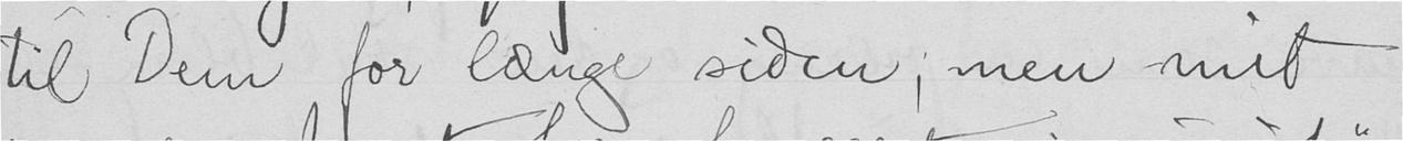til Dem for længe siden, men mit
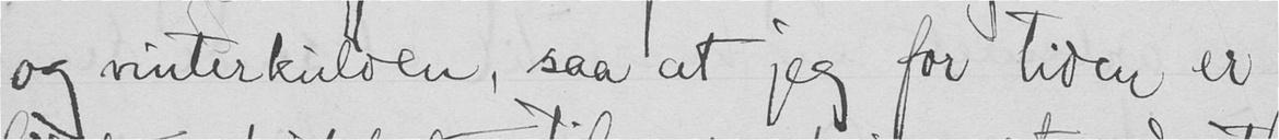og vinterkulden, saa at jeg for tiden er
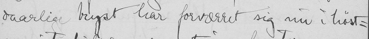daarlige bryst har forværret sig nu i høst-
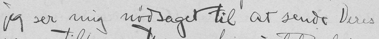jeg ser mig nødsaget til at sende Deres
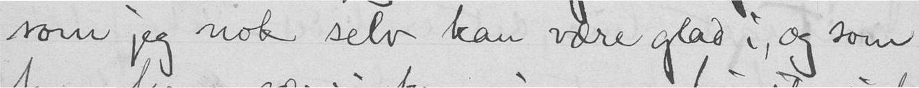som jeg nok selv kan være glad i, og som
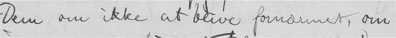Dem om ikke at blive fornærmet, om
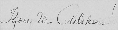Kjære Hr. Aslaksen!
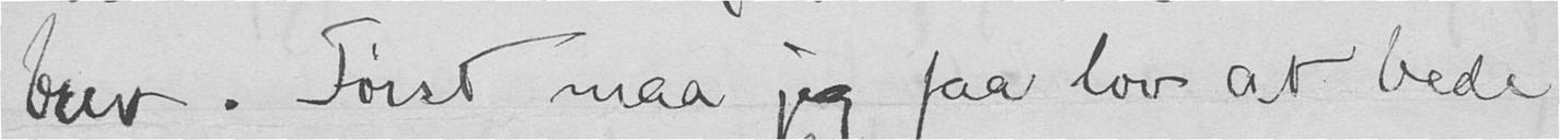brev. Først maa jeg faa lov at bede
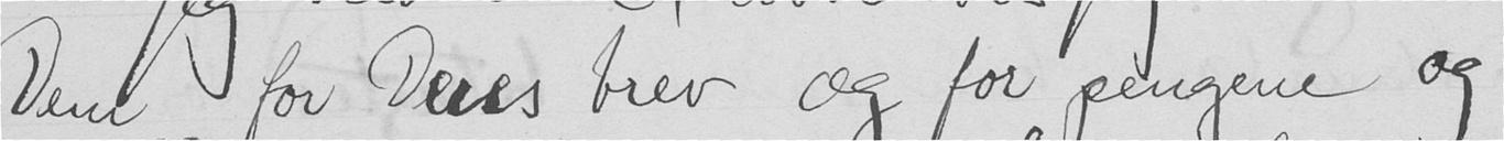Dem for Deres brev og for pengene og
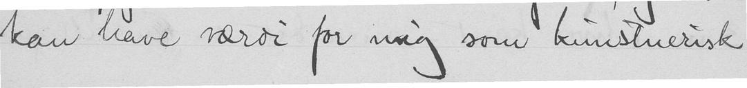kan have værdi for mig som kunstnerisk
